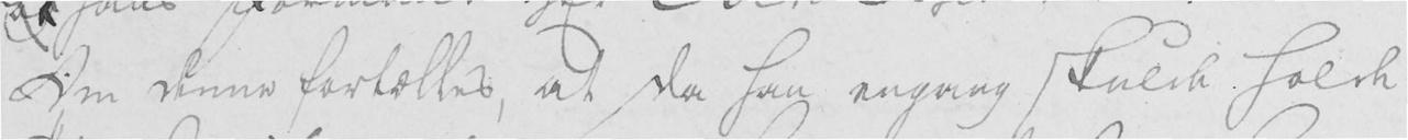Om denne fortælles, at da han engang skulde holde
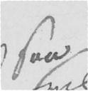saa
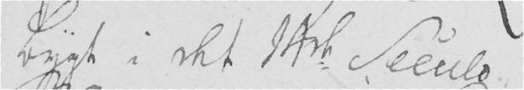bygt i det 14de Seculo
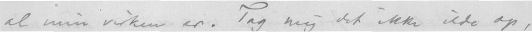al min virken er. Tag mig det ikke ilde op,
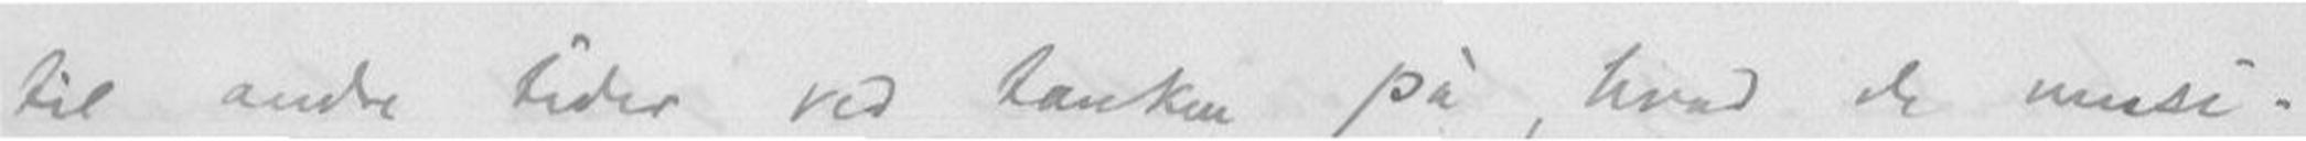til andre tider ved tanken på, hvad de musi-
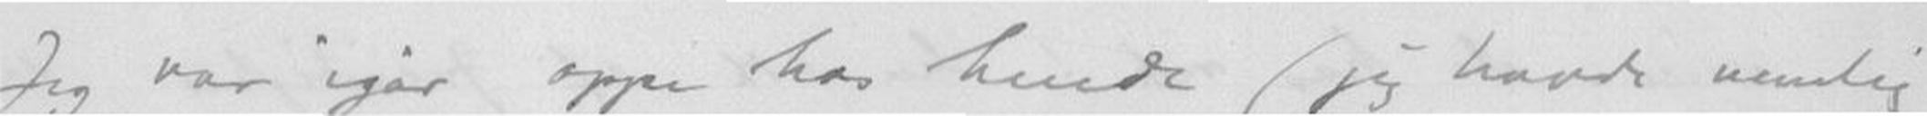Jeg var igår oppe hos hende (jeg havde nemlig
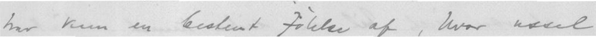har kun en bestemt følelse af, hvor ussel
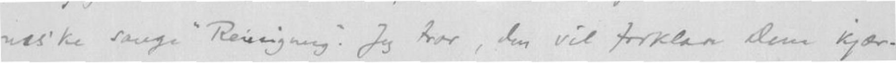næs'ke? sange "Reiesigning". Jeg tror, den vil forklare Dem kjær-
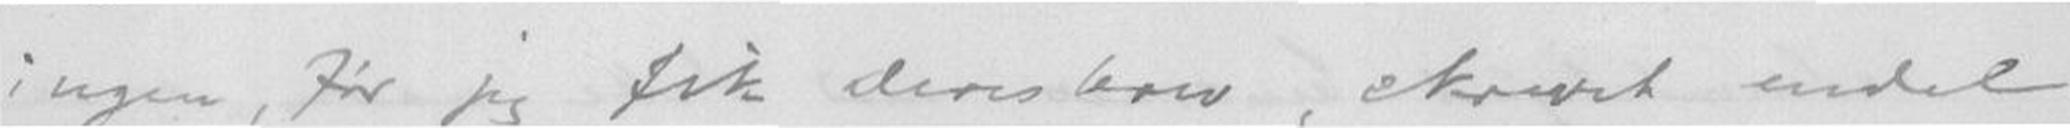ingen, før jeg fik Deres brev, skrevet endel
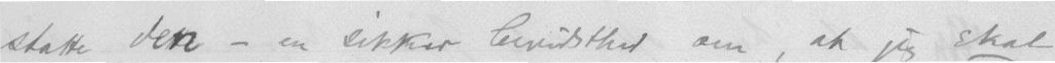statte den - en sikker bevidshed om, at jeg skal
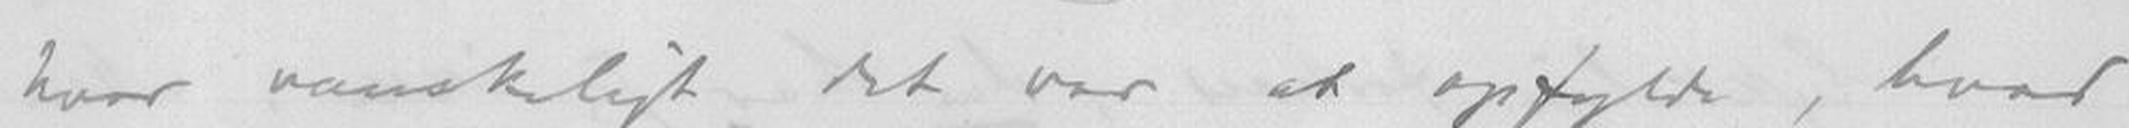hvor vanskeligt det var at opfylde, hvad
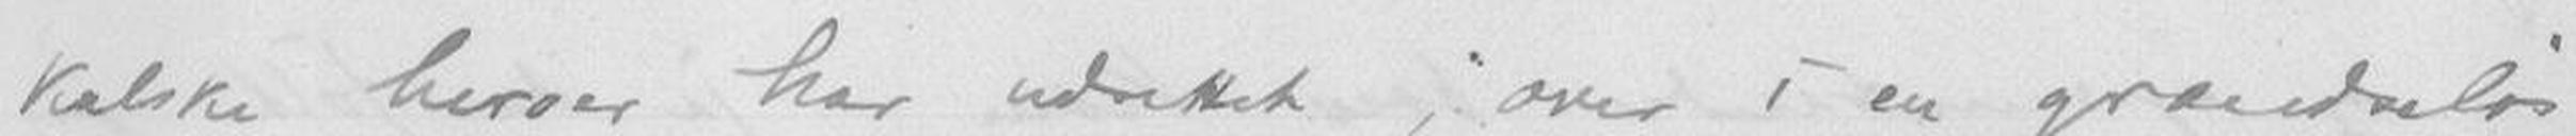kalske herrer har udrettet,; over i en grændseløs
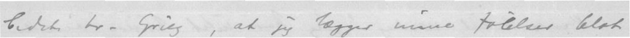bedste hr. Grieg, at jeg lægger mine følelser blot
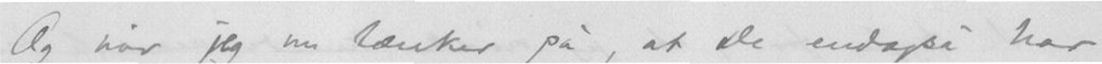Og når jeg nu tænker på, at De endogså har
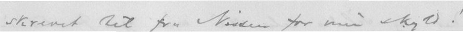skrevet til fru Nissen for min skyld!
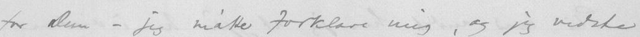for Dem - jeg måtte forklare mig, og jeg vidste
